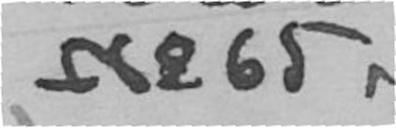No 65
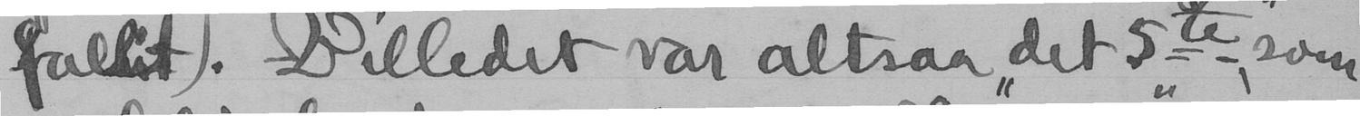fallit). Billedet var altsaa det 5te -, som
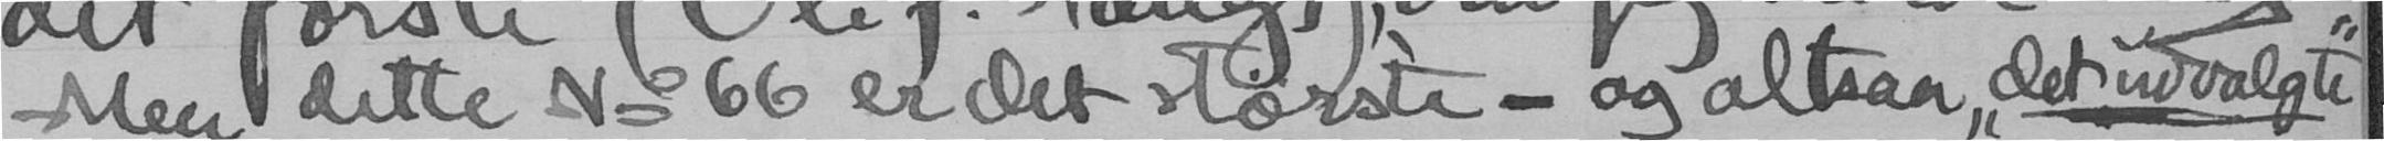Men dette No 66 er det største - og altsaa "det udvalgte"
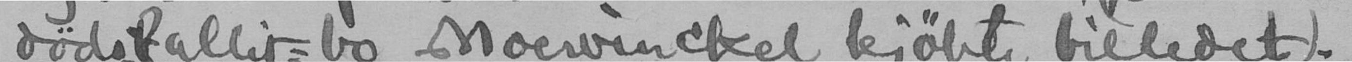dødsfallet - bo Moewinckel kjøbte billedet).
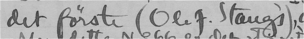det første (Ole J. Stangs),
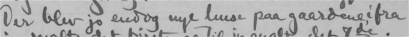Der blev jo endog nye huse paa gaardene i fra
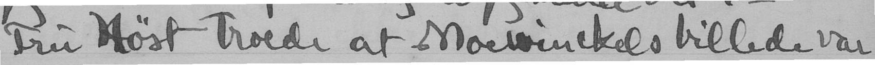Fru Høst troede at Moewinckels billede var
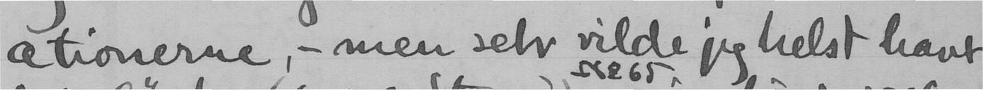ationerne - men selv vilde jeg helst havt
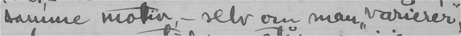samme motiv - selv om man "varierer"
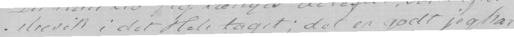Musik i det Hele taget; det er godt jeg har
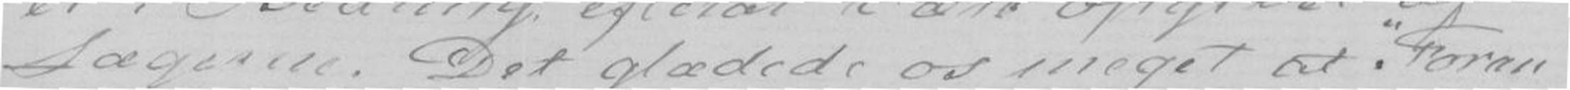Lægerne. det glædede os meget at "Foran
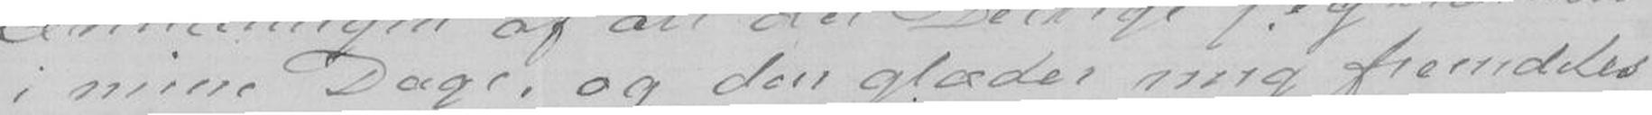i mine Dage, og den glæder mig fremdeles
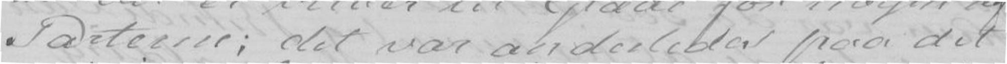Parterne; det var anderledes paa det
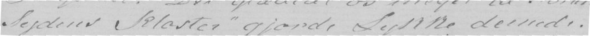Sydens Kloster! gjorde Lykke dernede.
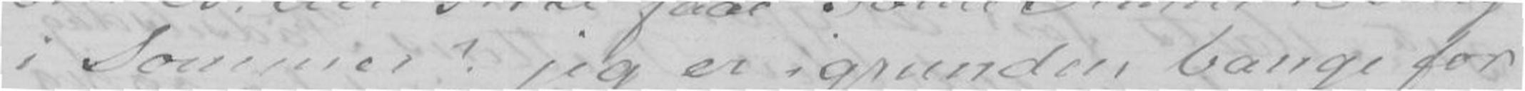i Sommer? jeg er igrunden bange for
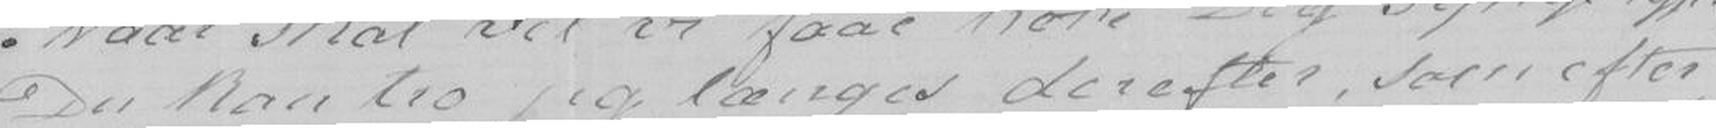Du kan tro jeg længes derefter, som efter
